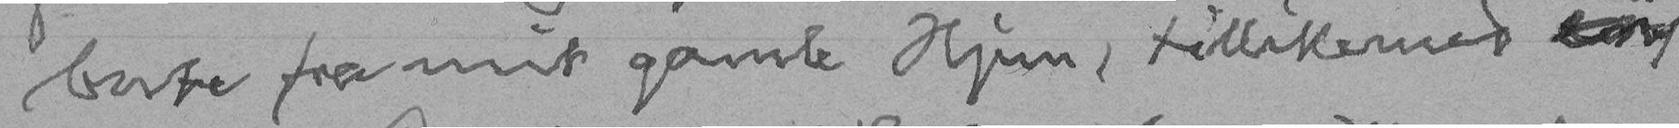borte fra mit gamle Hjem, tillikemed en
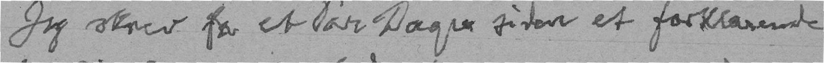Jeg skrev for et Par Dager siden et forklarende
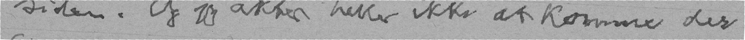siden. Og jeg akter heller ikke at komme der
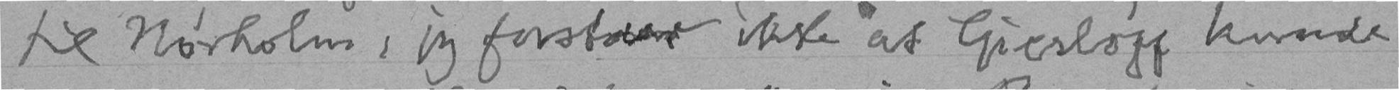til Nørholm, jeg forstaar ikke at Gierløff kunde
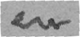en
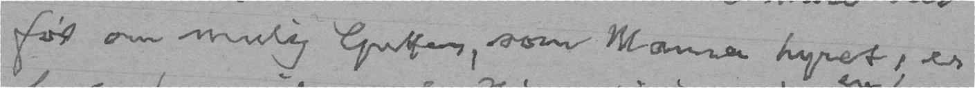før om mulig Gutten, som Mama hyret, er
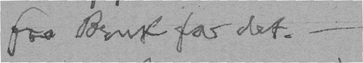faa Bruk for det. -
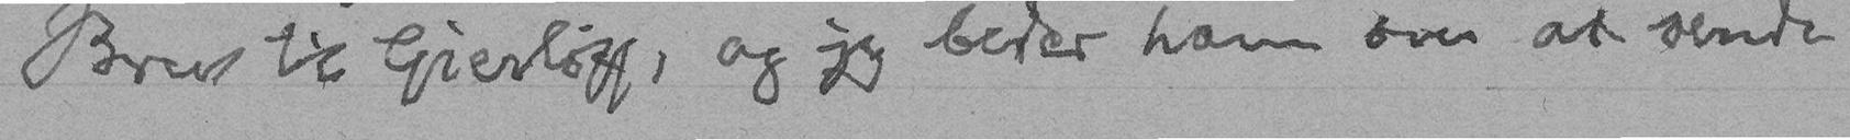Brev til Gierløff, og jeg beder ham om at sende
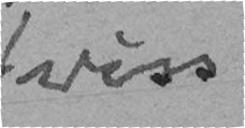viss
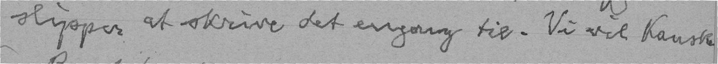slipper at skrive det engang til. Vi vil kanske
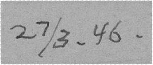27/3. 46.
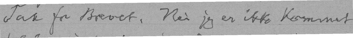Tak for Brevet. Nei jeg er ikke kommet
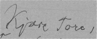Kjære Tore,
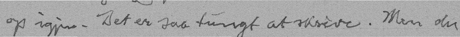op igjen. Det er saa tungt at skrive. Men du
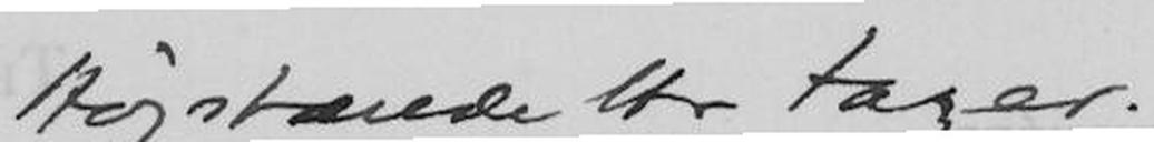Højstærede Hr Fazer.
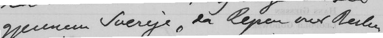gjennem Sverige, da Rejsen over Berlin
